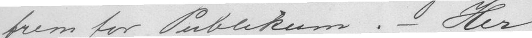frem for Publikum. - Her
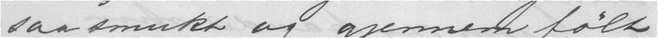saa smukt og gjennem følt,
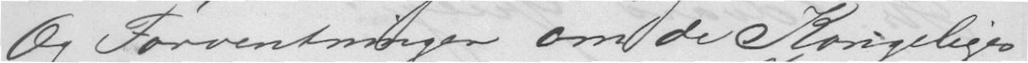Og Forventninger om de Kongeliges
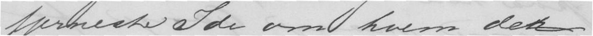fjerneste Ide om hvem der
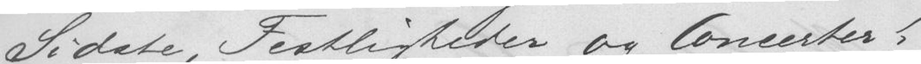Sidste, Festligheder og Concerter!
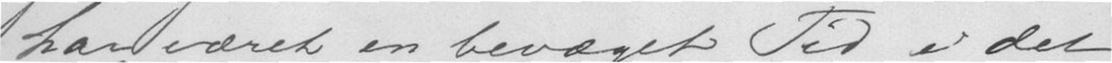har været en bevæget tid i det
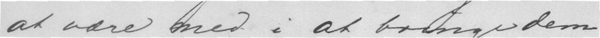at være med i at bringe dem
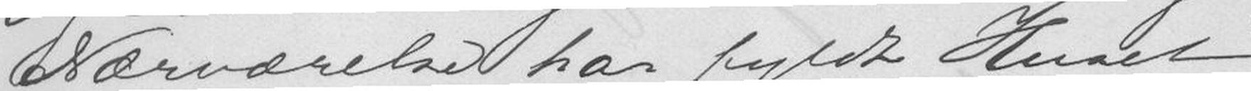Nærværelse har fyldt Huset
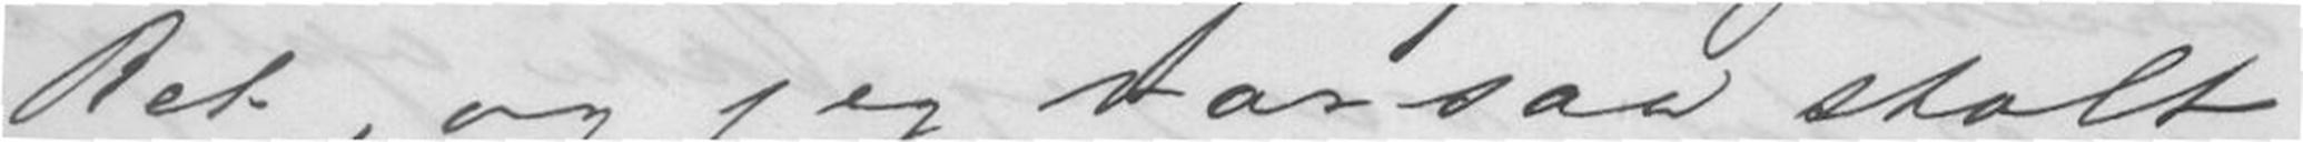Ret, og jeg var saa stolt
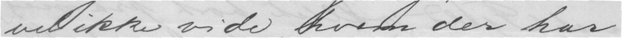vel ikke vide, hvem der har
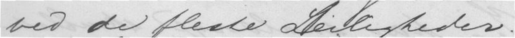ved de fleste Leiligheder.
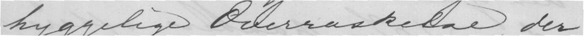hyggelige Overraskelse, der
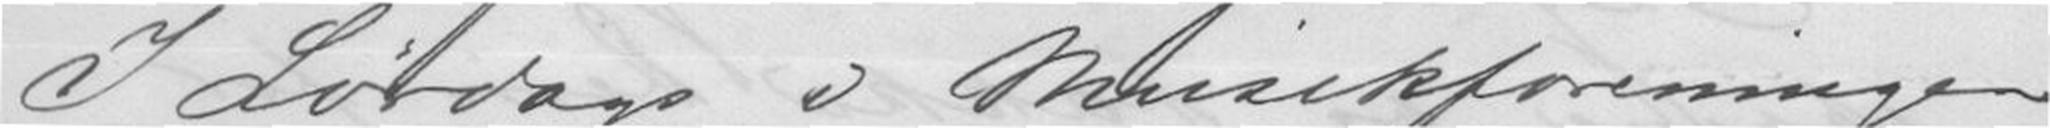I Lørdags i Musikforeningen,
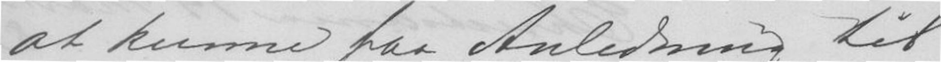at kunne faa Anledning til
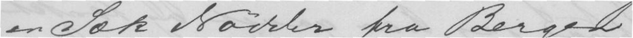en Sæk Nødder fra Bergen
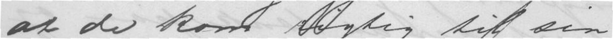at de kom riktig til sin
